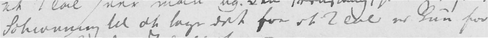Sohinnn til at lage det fra et 2 Tal er kun for
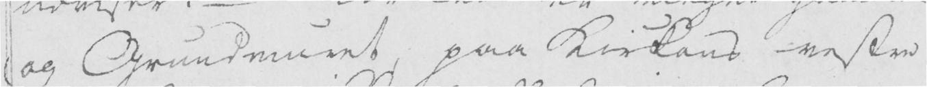og Grundmuret paa Kirkens vestre
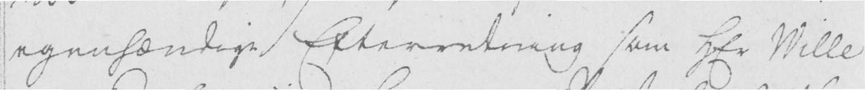egenhændige Efterretning som Hr Wille
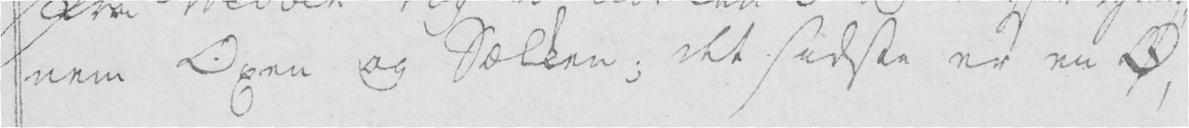nem Oxen og Sækken, det sidste er en Ø,
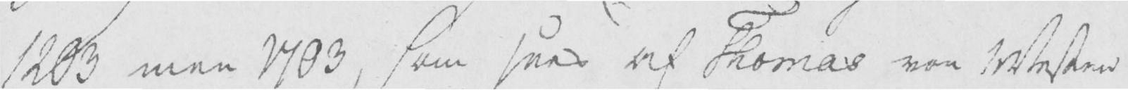1203 men 1703, som sies af Thomas von Westens
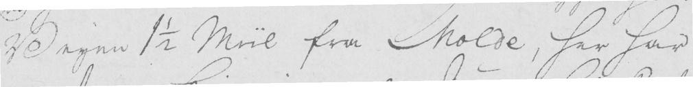Veyen 1/2 Miil fra Molde, her har
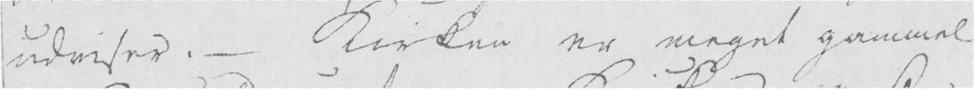udviser. - Kirken er meget gammel
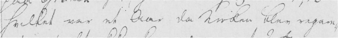hvilket var et Aar da Kirken blev repare-
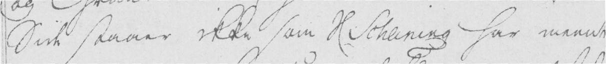Side staaer ikke som H. Schaning har meent
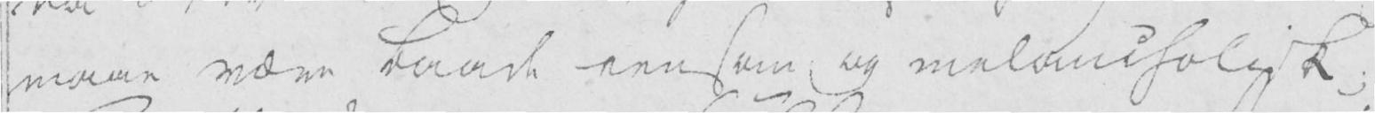maae være baade eensom og melancholisk;
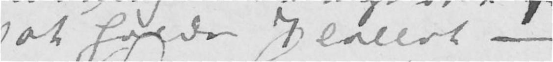at holde 7 Tallet -
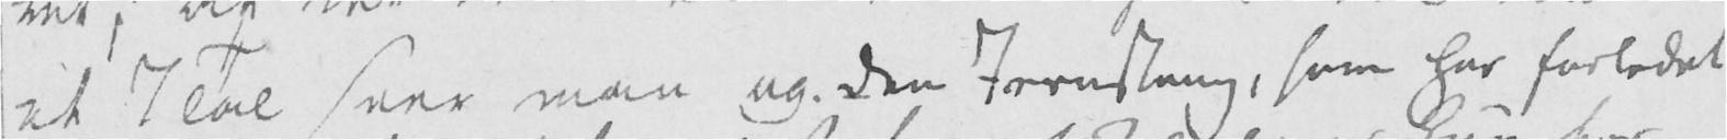et 7 Tal seer man og. Den Jernstang, som har forladet
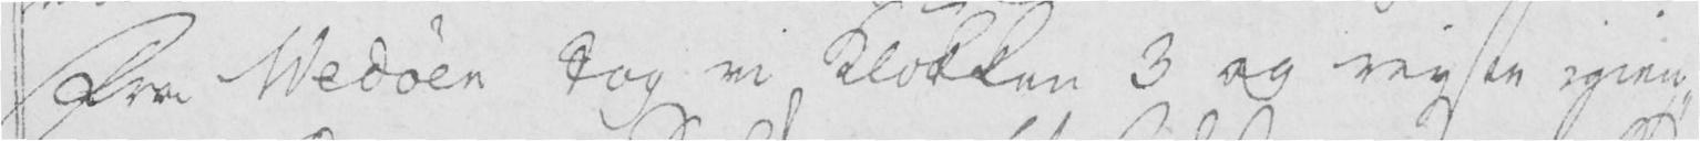Fra Wedøen tog vi Klokken 3 og reyste igien-
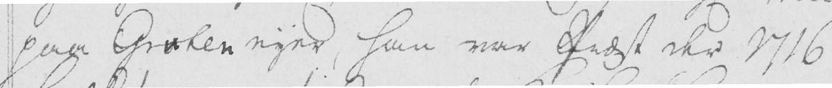paa Groten eyer, han var Præst der 1716
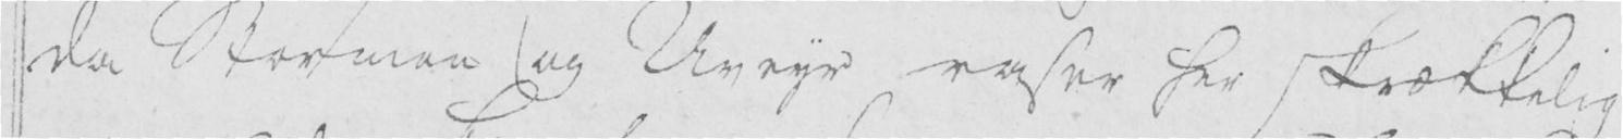da Stormer og Uveyr raser her skrækkelig
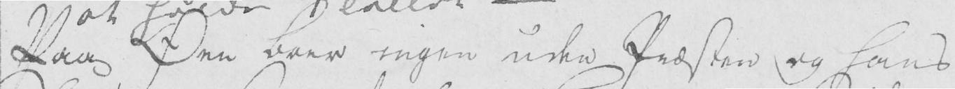Paa Øen boer ingen uden Præsten og hans
